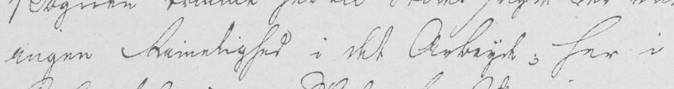ingen Rimelighed i det Arbeyde; her i
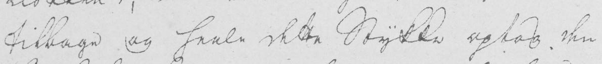tilbage og heele dette Stykke optog den
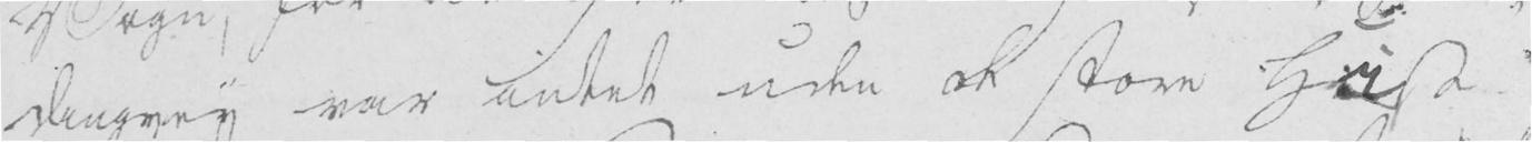dingvey var intet uden de Huse
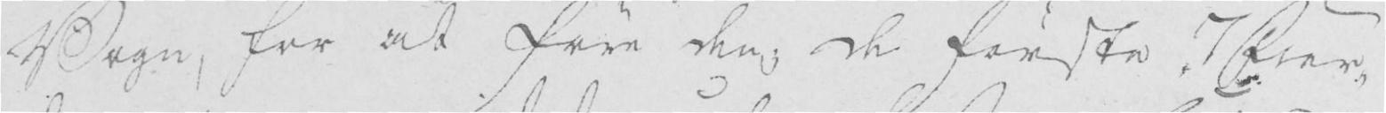Vogn, for at føre den; de første 7 Fier-
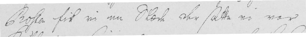Rosten fik vi nu Slæde der satte vi vor
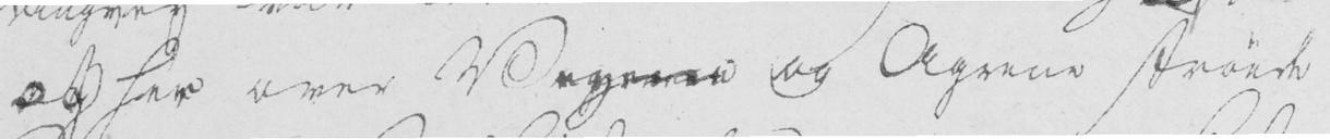og her over Veyerne og Agrene strøede
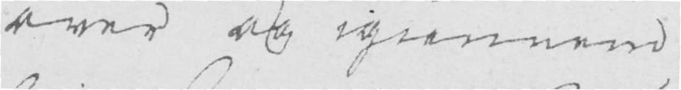over og igiennem
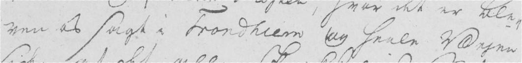ven os sagt i Trondhiem og heele Vejen
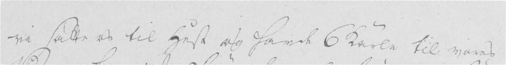vi satte os til Hest og havde 6 Karle til vores
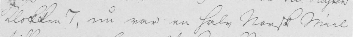Klokken 7, nu var en halv Norsk Miil
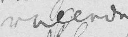rullede
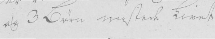og 3 Børn mistede Livet
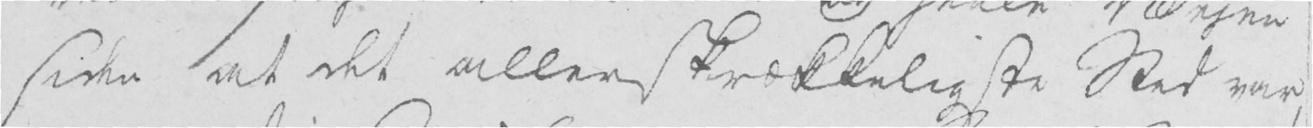siden at det allerskrækkeligste Sted var,
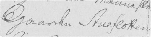Gaarden Stueflotten
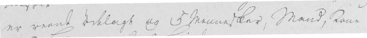er reent ødelagt og 5 Mennesker, Mand, Kone
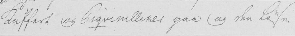Kuffert og Ciqvimlliner paa og den løse
